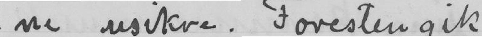ne usikre. Foresten gik
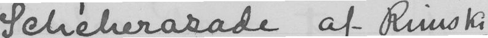Scheherazade af Rimski-
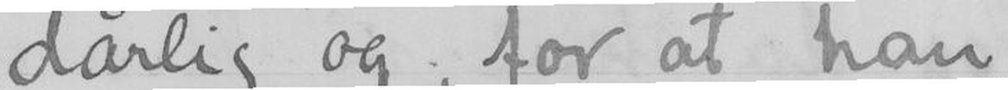dårlig og for at han
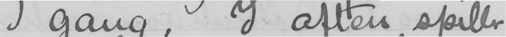1 gang. I aften spiller
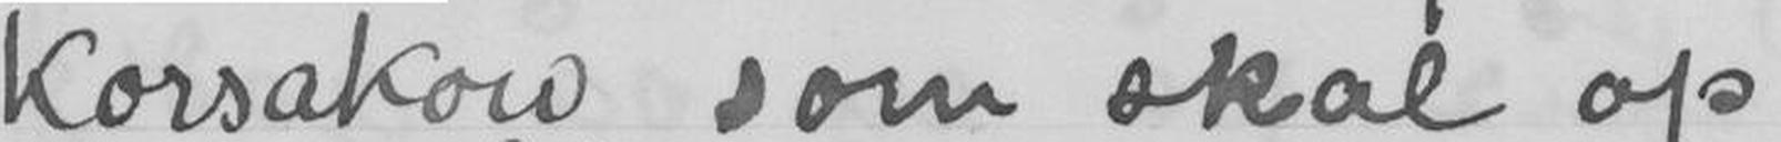Korsakow, som skal op
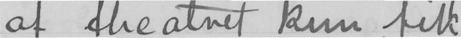af theatret kun fik
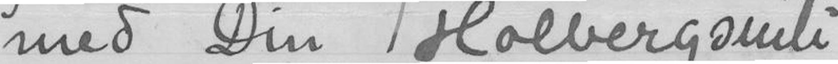med Din Holbergsuite
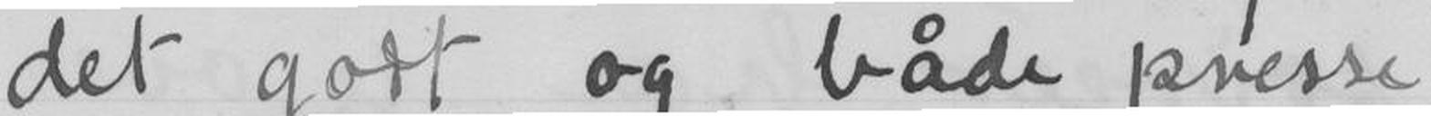det godt og både presse
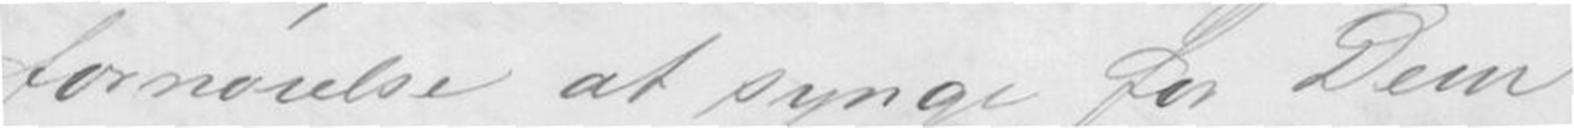fornøielse at synge for Dem
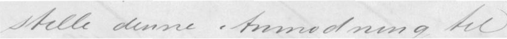stille denne Anmodning til
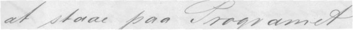at Staae paa Programmet
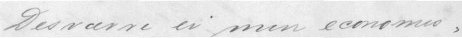Desværre er min economis-
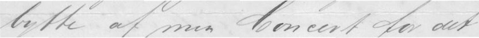bytte af min Concert for det
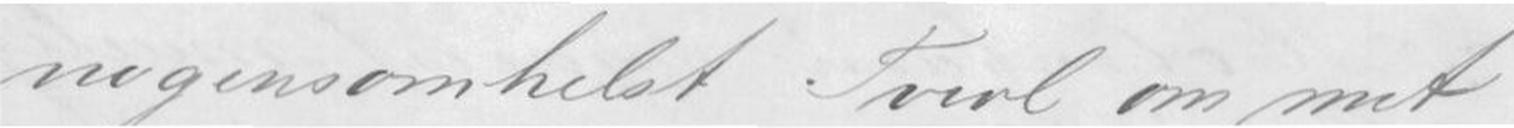nogensomhelst Tvivl om mit
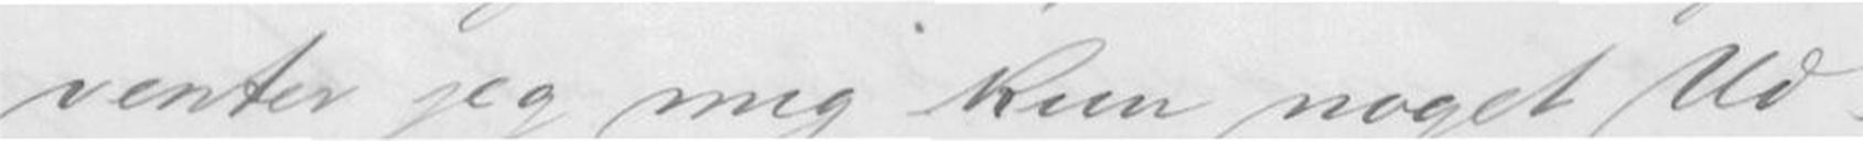venter jeg mig kun noget Ud-
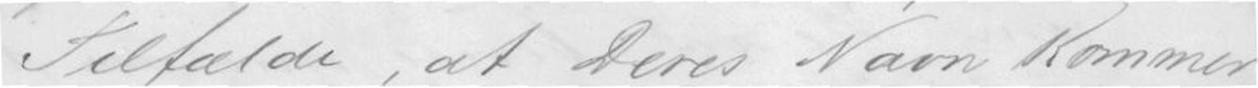Tilfælde, at Deres Navn kommer
